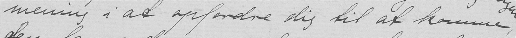mening i at opfordre dig til at komme
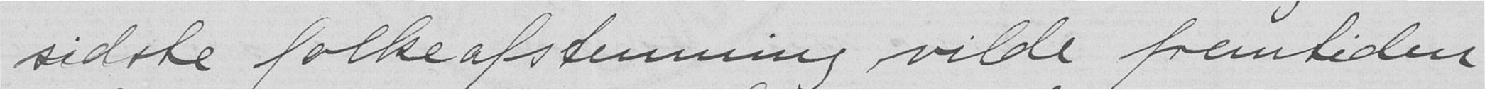sidste folkeafstemming vilde fremtiden
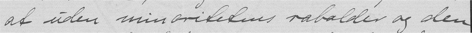at uden minoritetens rabalder og den
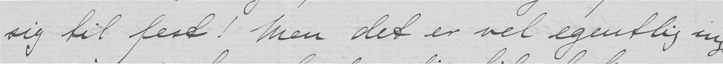sig til fest! Men det er vel egentlig ingen
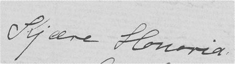Kjære Honoria!
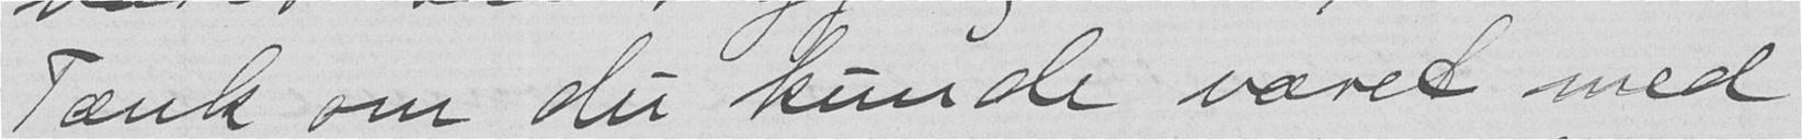Tænk om du kunde været med
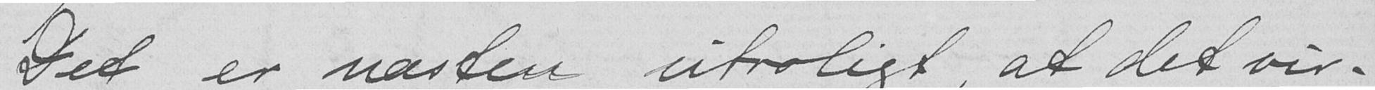Det er næsten utroligt, at det vir-
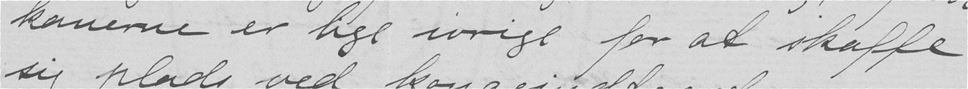kanerne er lige ivrige for at skaffe
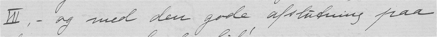VII, - og med den gode afslutning paa
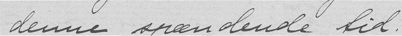denne spændende tid!
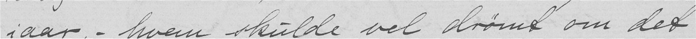iaar, - hvem skulde vel drømt om det
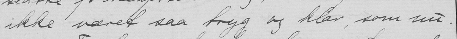ikke været saa tryg og klar, som nu.
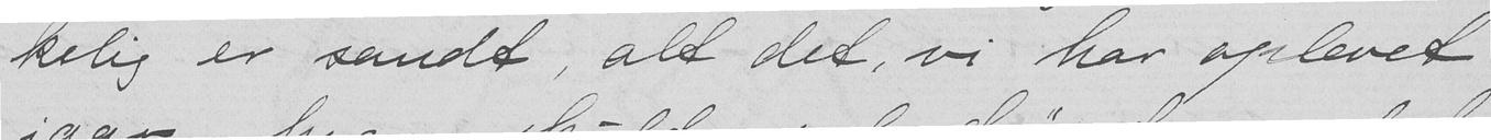kelig er sandt, alt det, vi har oplevet
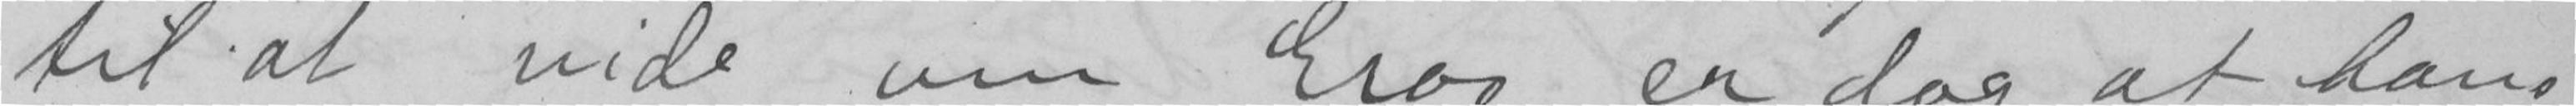til at vide om Eros er dog at hans
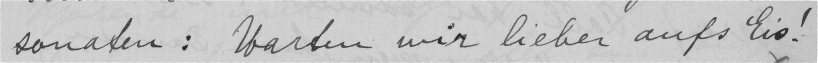sonaten: Warten wir lieber aufs Eis!
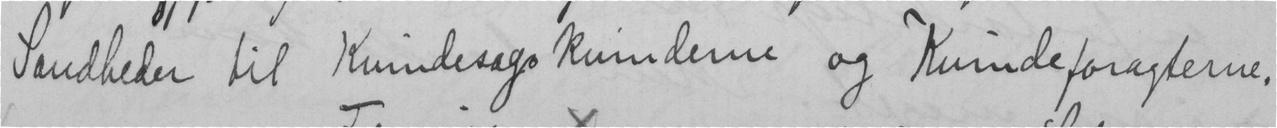Sandheder til Kvindesagskvinderne og Kvindeforagterne.
